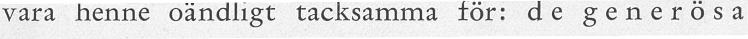vara henne oändligt tacksamma för: de generösa
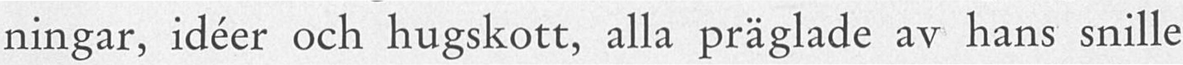ningar, idéer och hugskott, alla präglade av hans snille
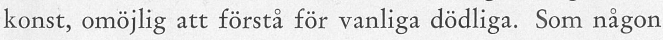konst, omöjlig att förstå för vanliga dödliga. Som någon
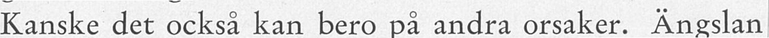Kanske det också kan bero på andra orsaker. Ängslan
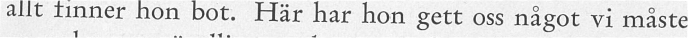allt finner hon bot. Här har hon gett oss något vi måste
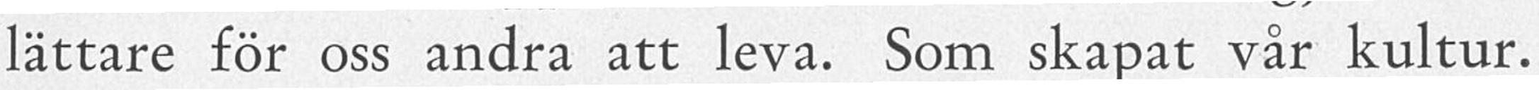lättare för oss andra att leva. Som skapat vår kultur.
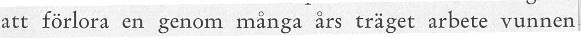att förlora en genom många års träget arbete vunnen
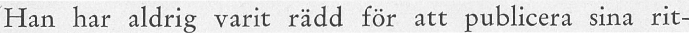Han har aldrig varit rädd för att publicera sina rit-
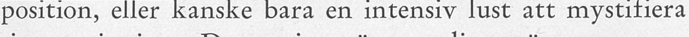position, eller kanske bara en intensiv lust att mystifiera
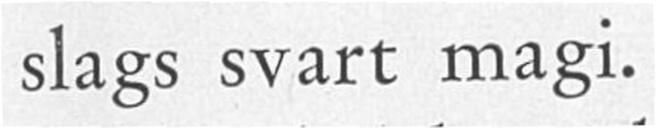slags svart magi.
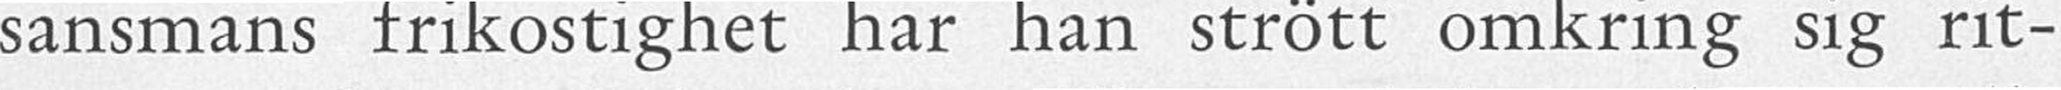sansmans frikostighet har han strött omkring sig rit-
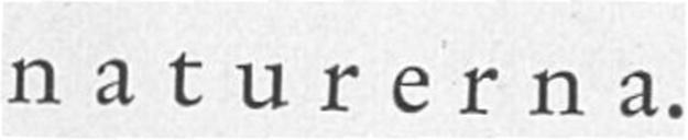naturerna.
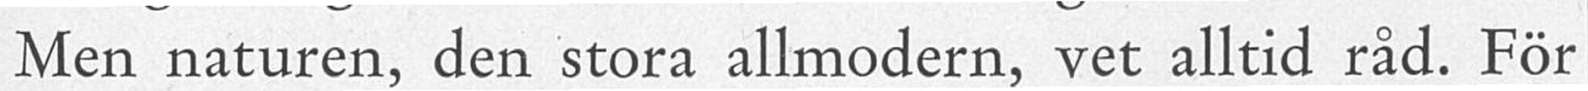Men naturen, den stora allmodern, vet alltid råd. För
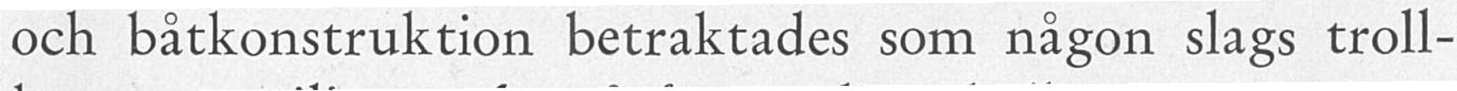och båtkonstruktion betraktades som någon slags troll-
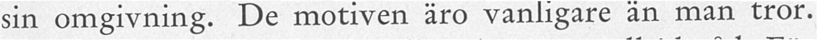sin omgivning. De motiven äro vanligare än man tror.
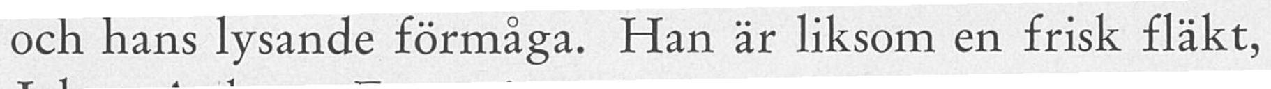och hans lysande förmåga. Han är liksom en frisk fläkt,
